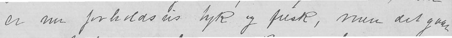er nu forholdsvis tyk og frisk, men det gaar
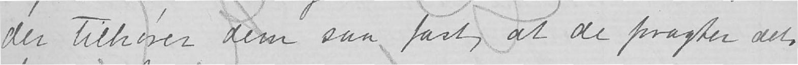der tilhører dem saa fast, at de foragter det.
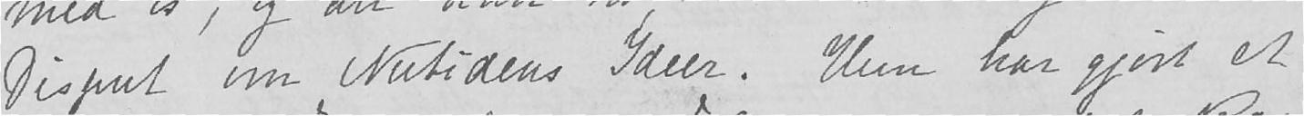Disput om Nutidens Ideer. Hun har gjort et
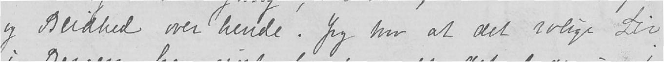og Blidhed over hende. Jeg tror at det rolige Liv
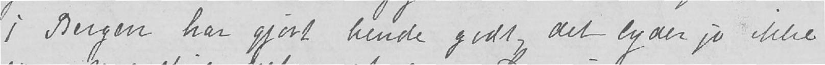i Bergen har gjort hende godt, det lyder jo ikke
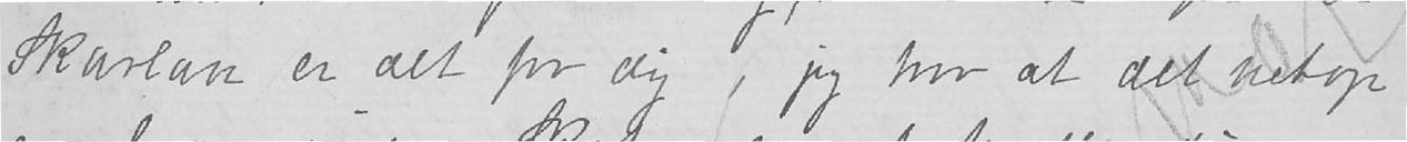Skavlan er alt for dig, jeg tror at det netop
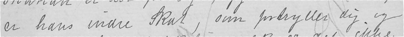er hans indre Skat, som fortryller dig og
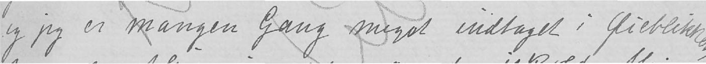og jeg er mangen Gang meget indtaget i Øieblikket,
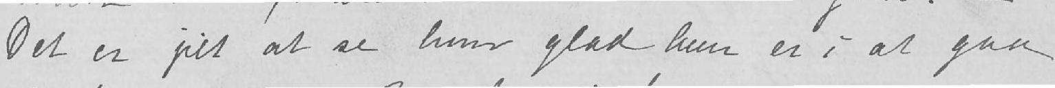Det er jilt at se hvor glad hun er i at gaa
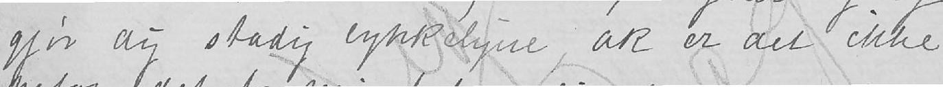gjør dig stadig lykkeligere, ak er det ikke
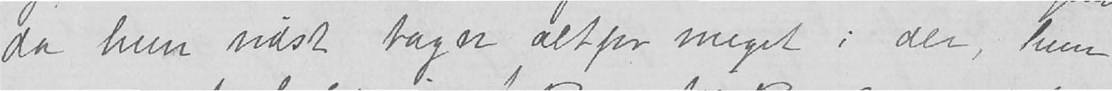da hun vidst tager altfor meget i der, hun
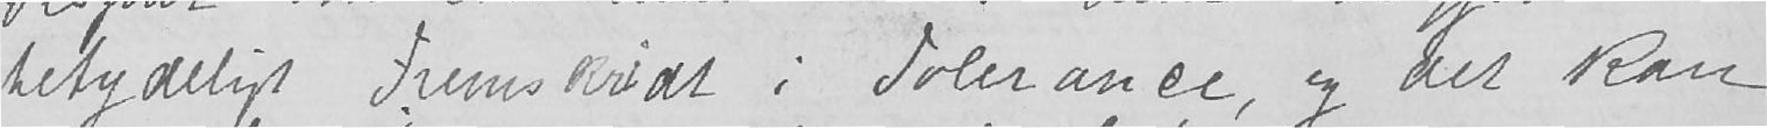betydeligt Fremskridt i Tolerance, og det kan
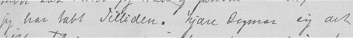jeg har tabt Tilliden. Kjære Dagmar sig det
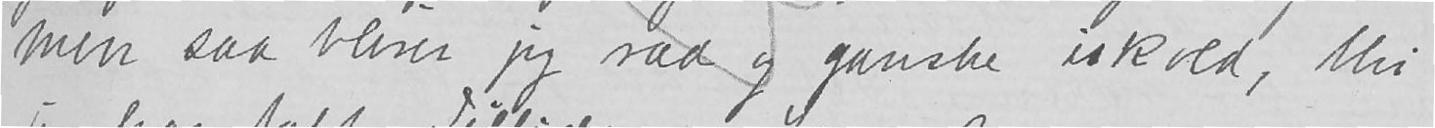men saa bliver jeg ræd og ganske iskold, thi
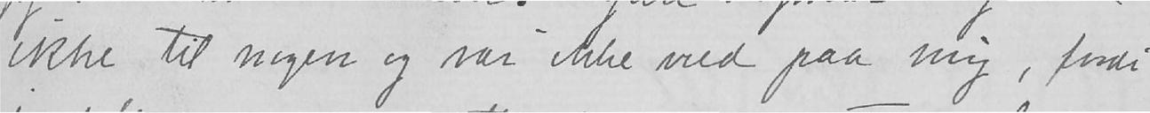ikke til nogen og vær ikke vred paa mig, fordi
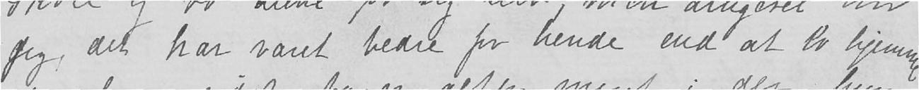jeg, det har været bedre for hende end at bo hjemme,
